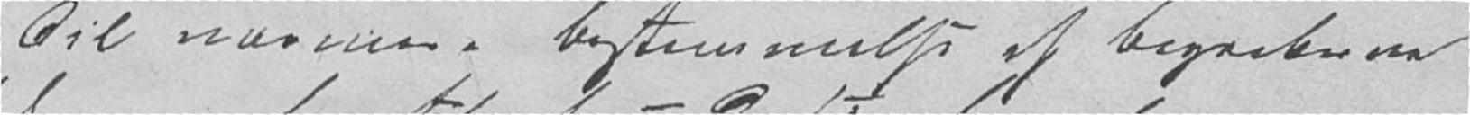Til nærmere Bestemmelse af begreberne
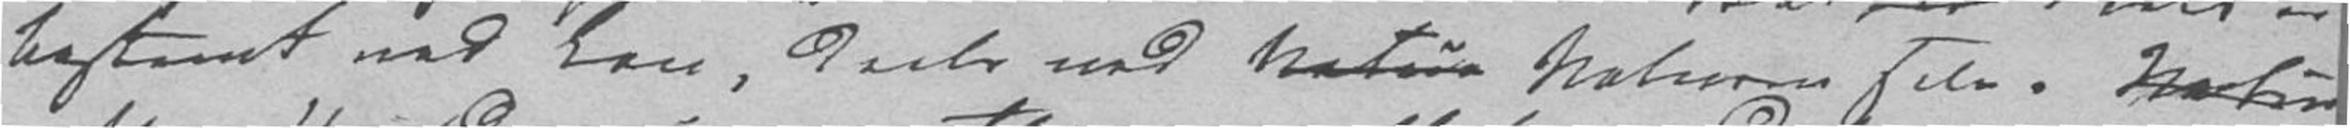bestemt ved Lov, deels ved Naturen selv. Natur
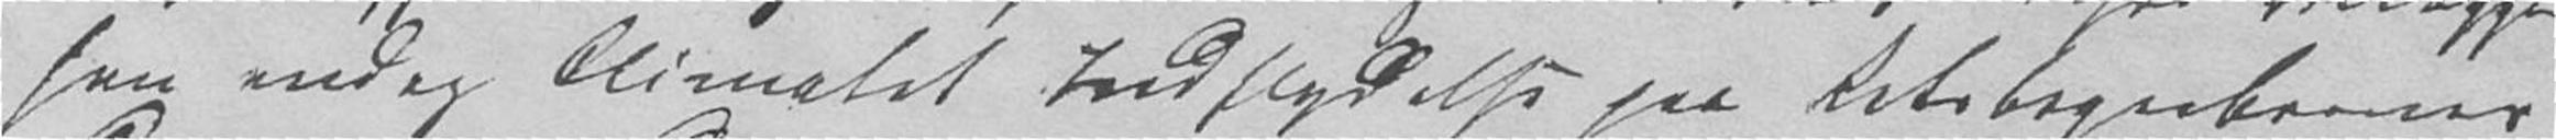han endog Climatet Indflydelse paa Retsbegrebernes
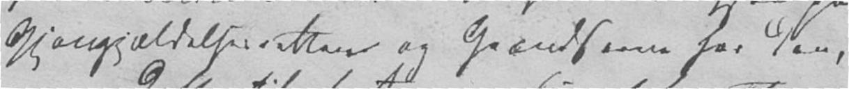Gjengjældelsesretten og Grændserne for den,
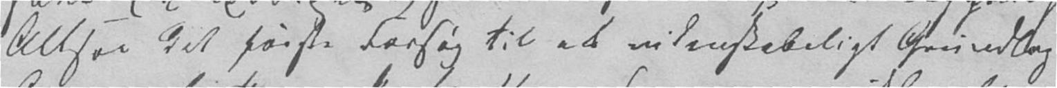Altsaa det første Forsøg til et videnskabeligt Grundlag
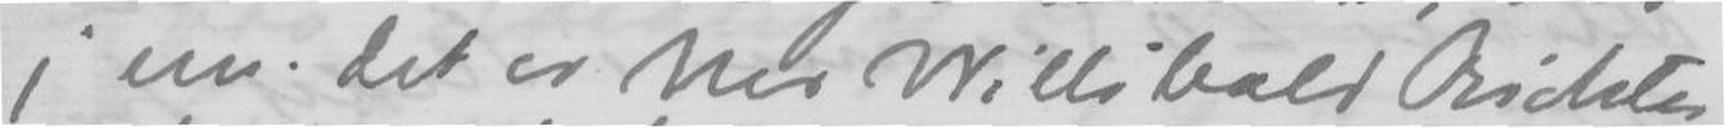j nu det er Mr Willibald Richter
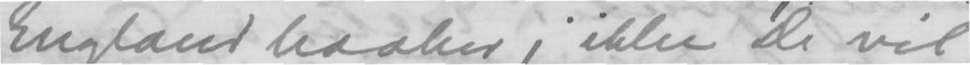England haaber j ikke De vil
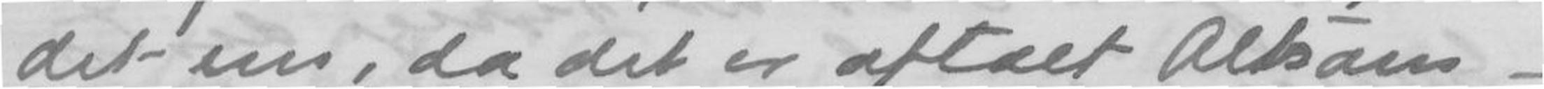det nu, da det er aftalt Altsam-
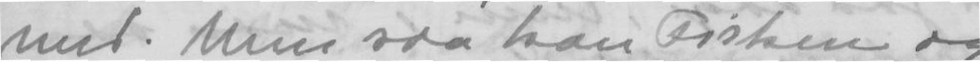med. Men saa kan Fisken og
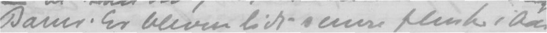Baner. Er bleven lidt mere flink i Aar
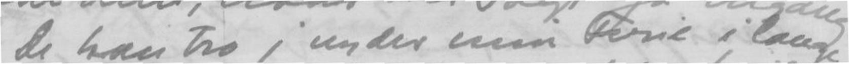- De kan tro j nyder min Ferie i lange
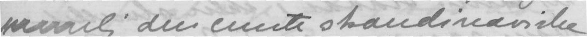nemlig den eneste skandinaviske
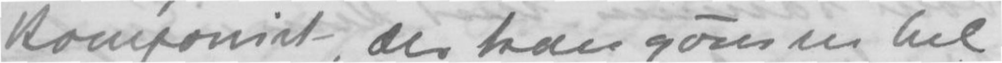Komponist der kan gøres en hel
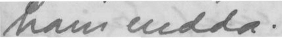ham endda.
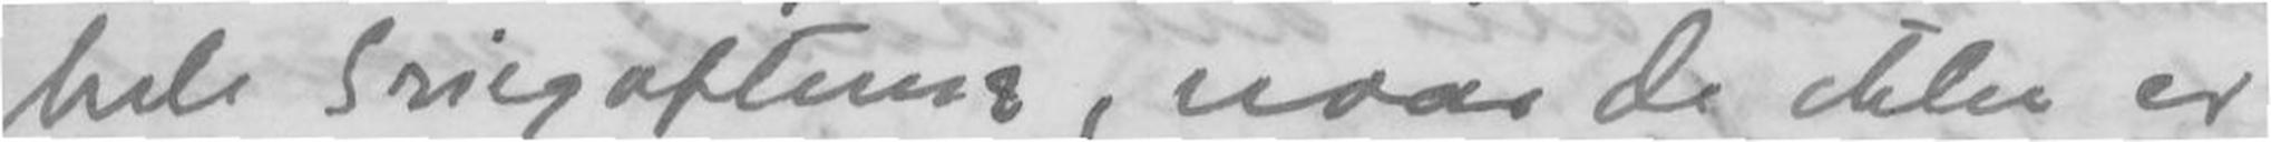hele Griegaftener, naar De ikke er
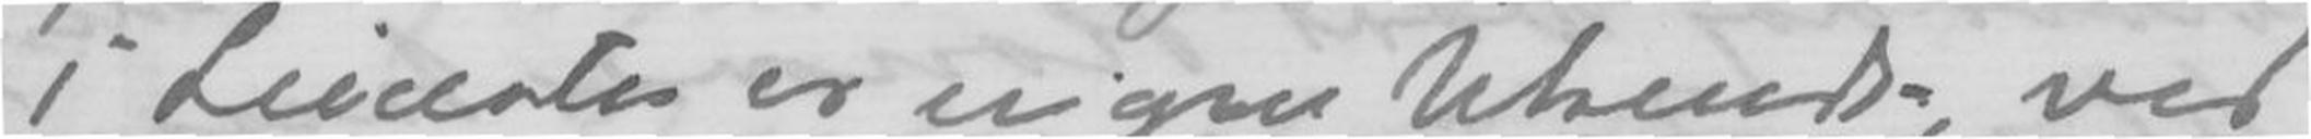i Leicester er ingen Ukendt, ved
